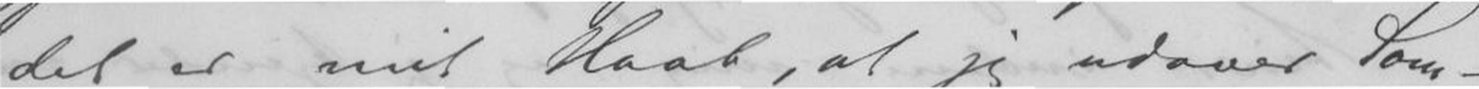det er mit Haab, at jeg udover Som-
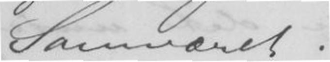Samværet.
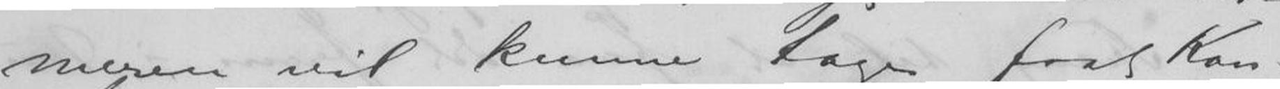meren vil kunne tage fast Kon-
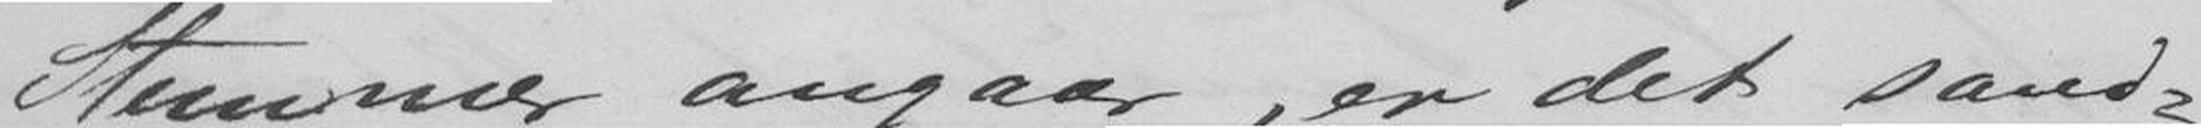Stemmer angaar, er det sand-
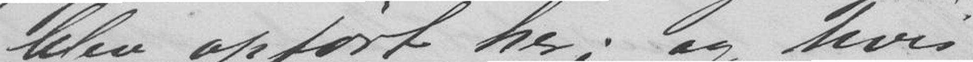blev opført her; og hvis
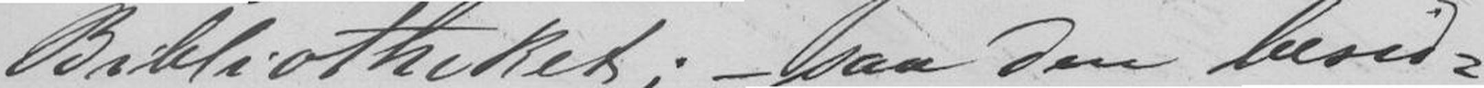Bibliotheket; - saa den besid-
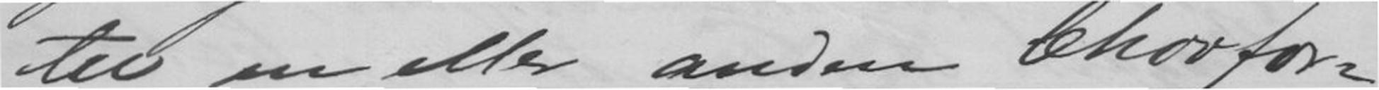til en eller anden Chorfor-
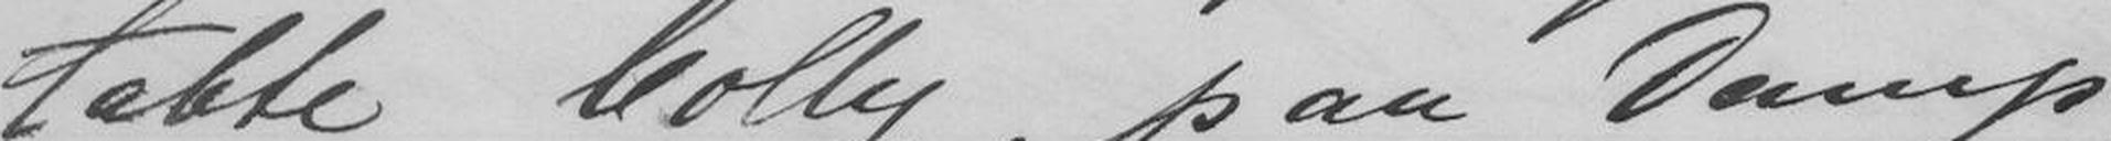tabte Colly, paa Damp
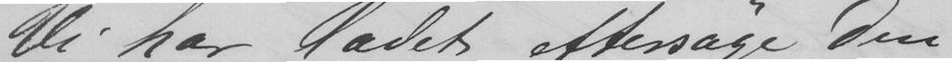Vi har ladet eftersøge Din
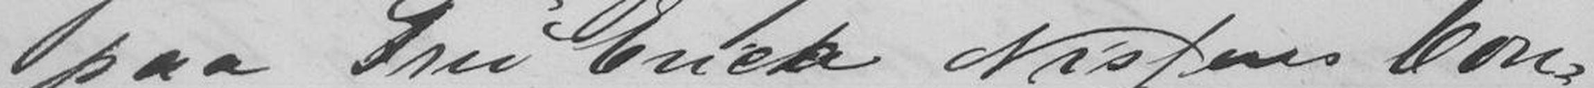paa Fru Erica Nissens Con-
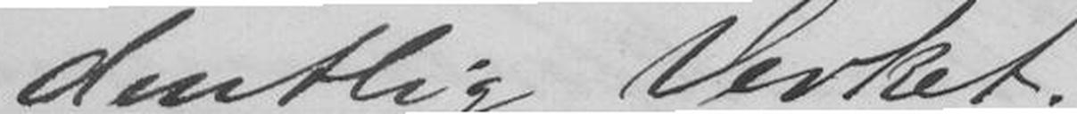dentlig Verket.
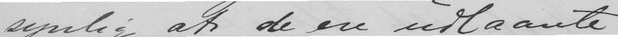synlig at de ere udlaante
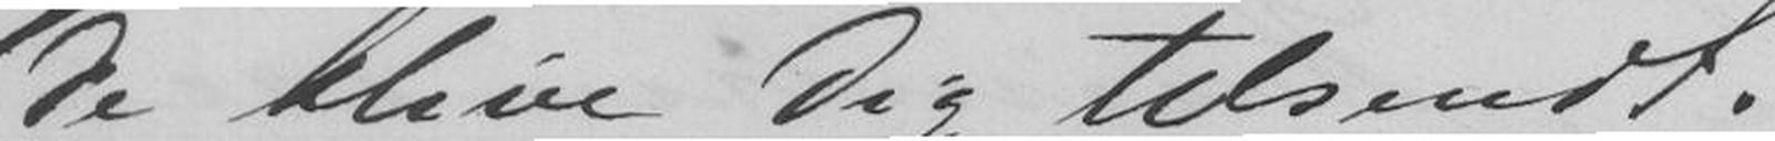De blive Dig tilsendt.
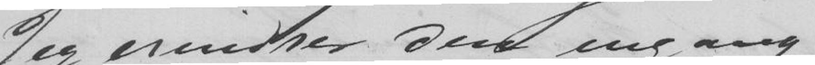Jeg erindrer den engang
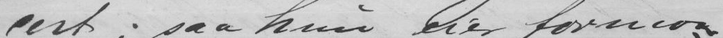cert; saa hun eier formo-
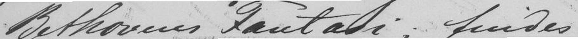Bethovens Fantasi, findes
